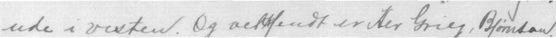ude i Vesten. Og velkjendt er Her Grieg, Bjørnson,
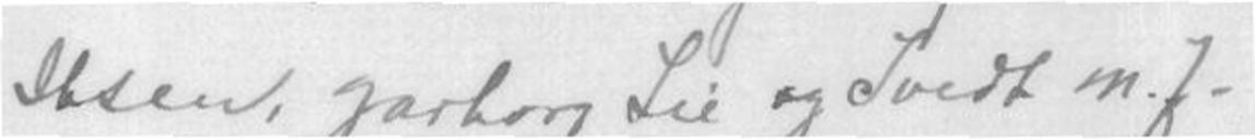Ibsen, Garborg Lie og Tvedt m.f.
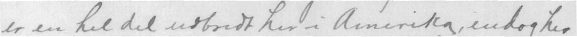er en hel del udbredt her i Amerika, endog her
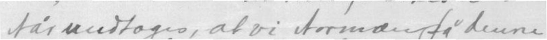Når undtages, at vi Normæn på denne
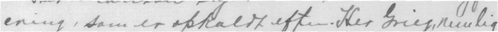ening, som er opkaldt efter Her Grieg, nemlig
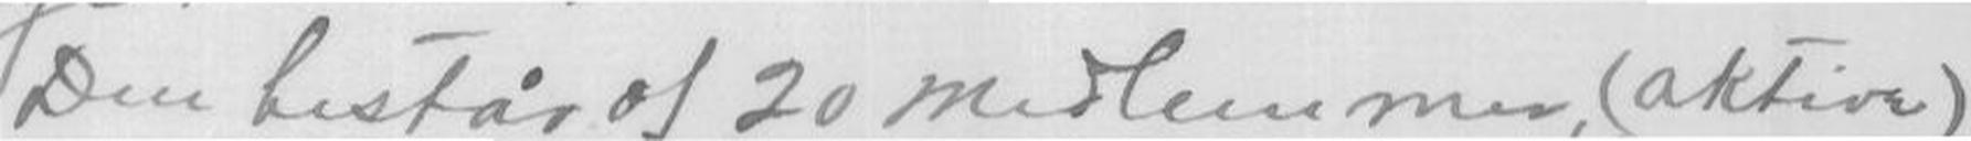Den består af 20 Medlemmer (aktive)
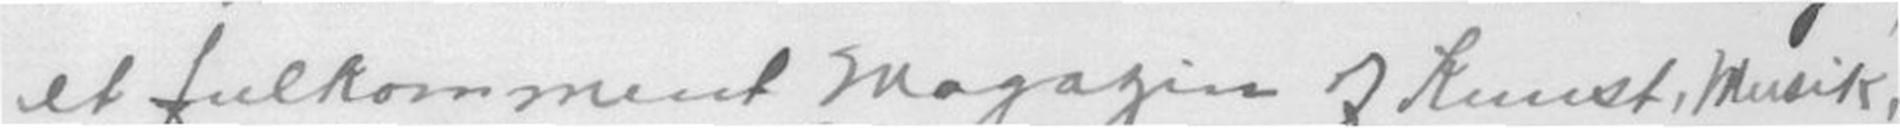et fulkomment Magazin of Kunst, Musik,
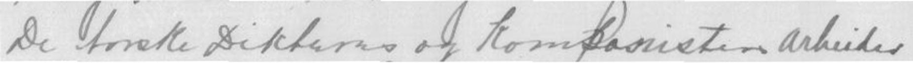De Norske Digteres og Komponisters Arbeider
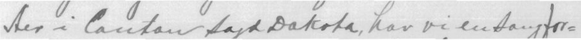Her i Canton Sajd Dakota, har vi en Sangfor-
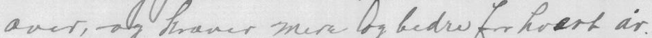over, og kræver mere og bedre for hvært År.
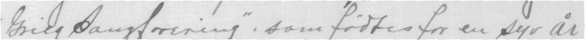"Grieg Sangforening", som fødtes for en syv År
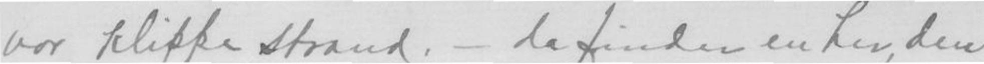vor klippe Strand, - da finder en her, den
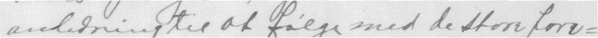anledning til at følge med de store fore-
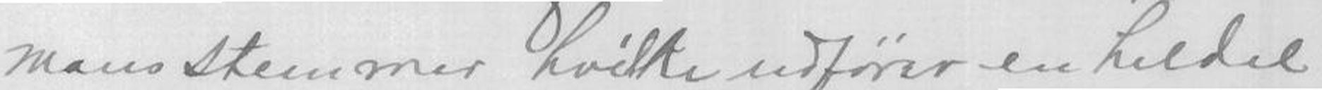Mansstemmer hvilke udfører en heldel
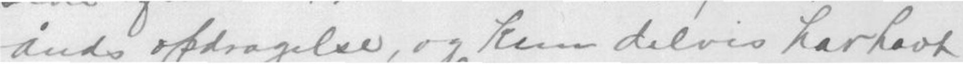Ånds opdragelse, og kun delvis har havt
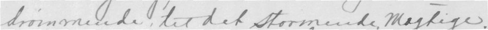drømmende, let det stormende, Mægtige.
